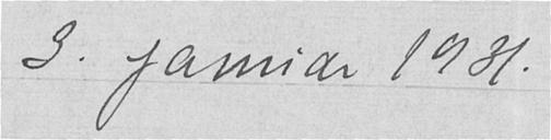3. januar 1931.
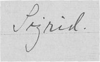Sigrid
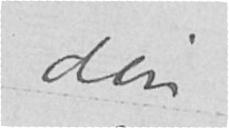din
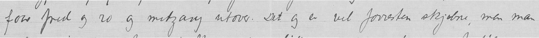faar fred og ro og medgang utover. Det og er vel forresten skjæbne, men man
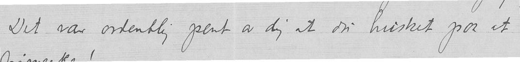Det var ordentlig pent av dig at du husket paa at
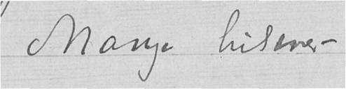Mange hilsener
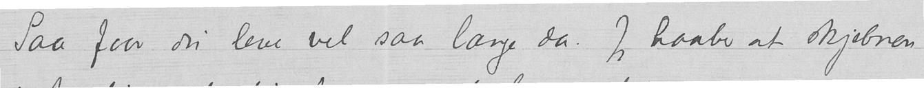Saa faar du leve vel saa længe da. Jeg haaber at skjebnen
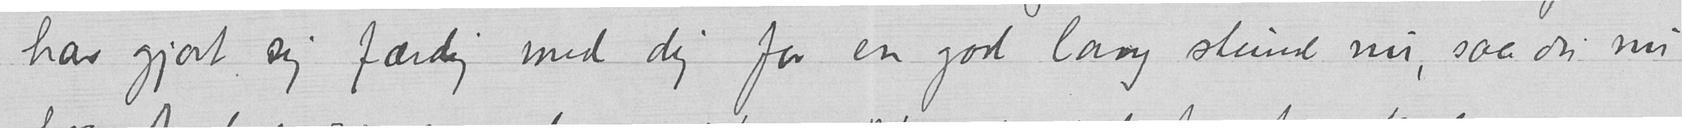har gjort sig færdig med dig for en god lang stund nu, saa du nu
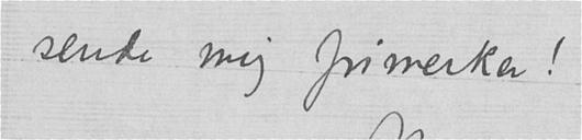sende mig frimerker!
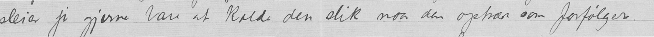pleier jo gjerne bare at kalde den slik naar den optrær som forfølger.
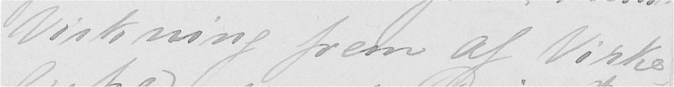Virkning frem af Virke-
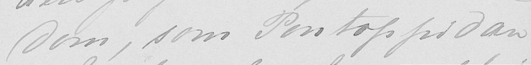dom, som Pontoppidan
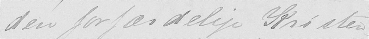den forfærdelige Kristen-
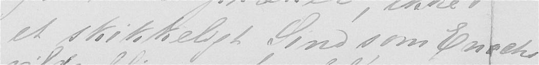et skikkeligt Sind som Enoch
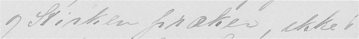og Kirken præker, ikke
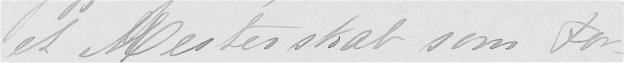et Mesterskab som For-
